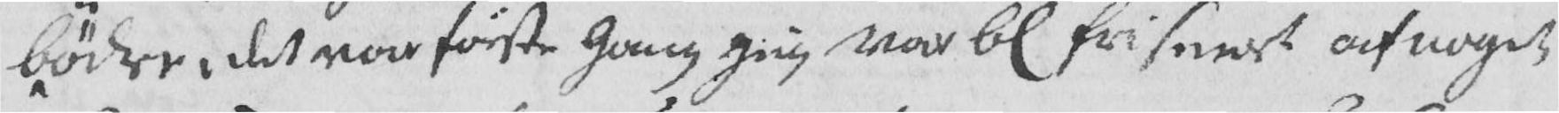bødre, det var første gang jeg var bl friseret af noget
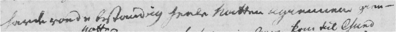havde roede bestandig heele Natten igiennem gien-
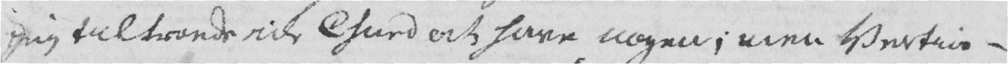jeg tiltroede icke Csund at have nogen; men Vertin-
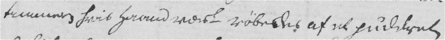timmers hvis Haandværk røbedes af et pudderet
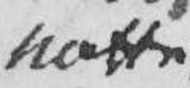Natte
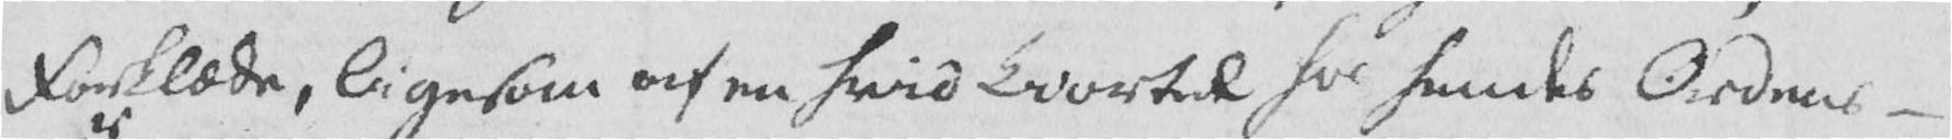Forklæde, ligesom af en hvid Kiortel hos hendes Ordens-
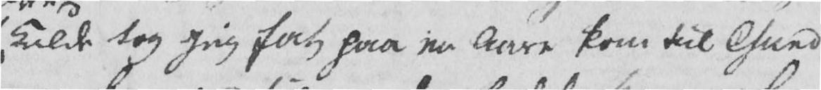Kulde tog jeg fat paa en Aare kom til Csund
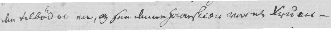den tilbød os en, og see denne Haarskiær var et Fruen-
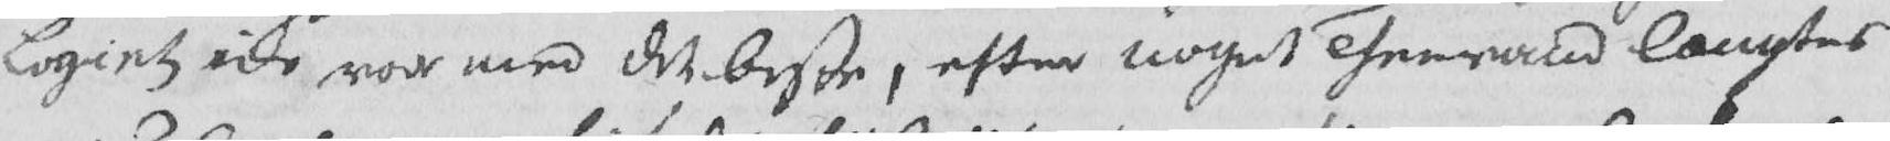Logiet icke var med det beste, efter noget Theevand længtes
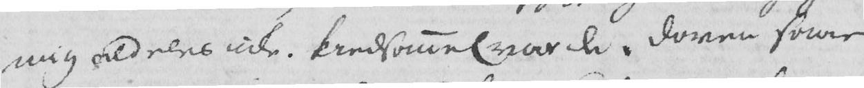mig aldeles icke. kiedsomel var de, dovne saae
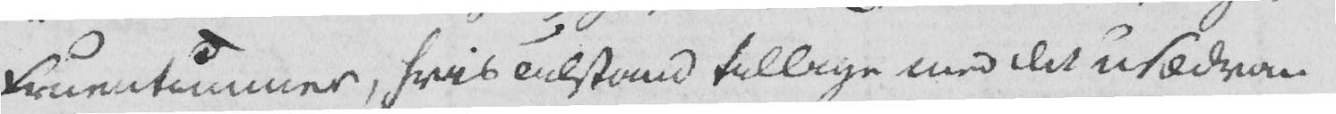Fruentimmer, hvis Tilstand tillige med det usædvan-
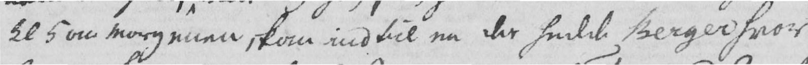Kl 5 om Morgenen, kom ind til en der hedde Berger hvor
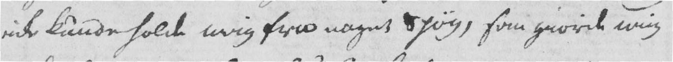icke kunde holde mig fra noget Spøg, som giorde mig
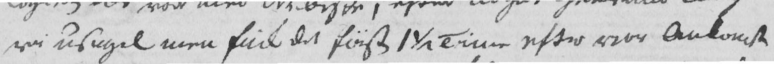vi usigel men fik det først 1 1/2 Time efter vor Ankomst
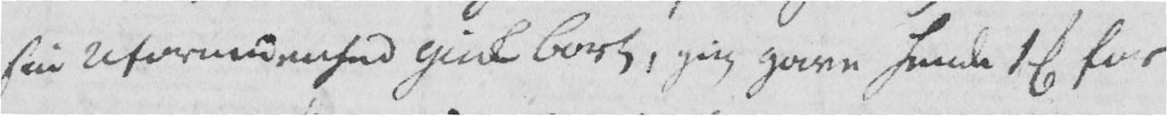sin Uformuenhed gik bort, jeg gave hende 1 sk for
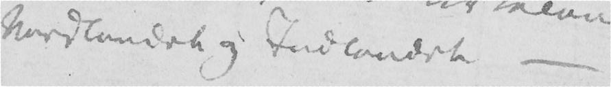Nordlandet og Indlandet -
